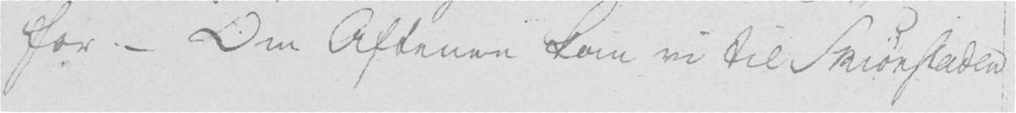for. - Om Aftenen kom vi til Skiønstaden
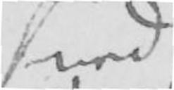ned
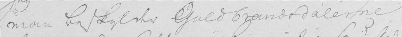man beskylder Guldbrandsdølerne
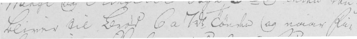bliver til Brød 6 a 7 rd Tønder og naar Fi-
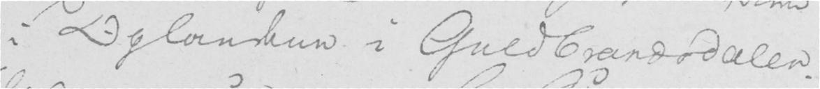i Oplandene i Guldbrandsdalen
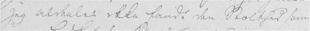jeg aldeeles ikke fandt den Stolthed som
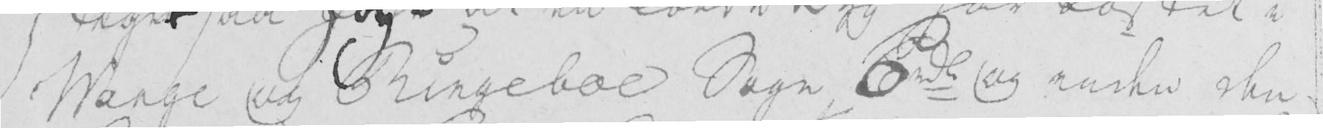Wange og Ringeboe Sogn 6 rde og anden den
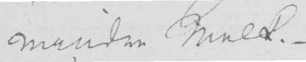mindre Melk. -
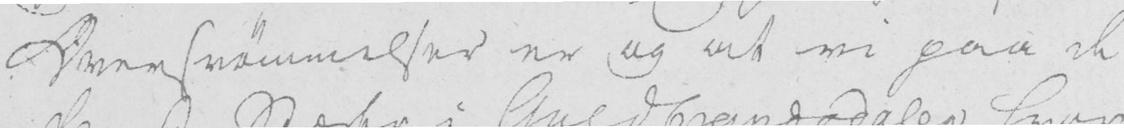Oversvømmelser er og at vi paa de
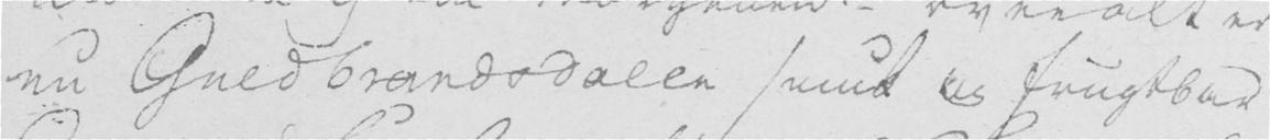nu Guldbrandsdalen smuk og frugtbar
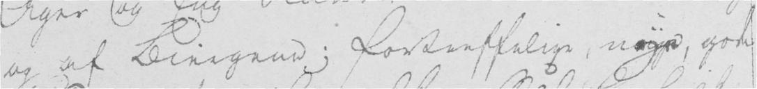og af Biergene; fortreffelige, nøye, gode
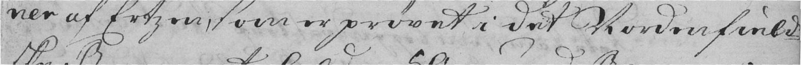ner af Ertzen, som er prøvet i det Nordenfield-
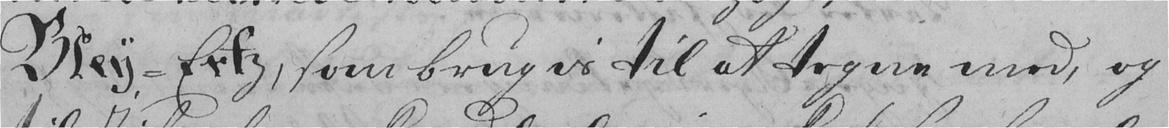Bley-Ertz, som brugis til at tegne med, og
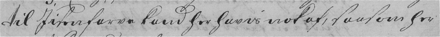til Iisenfarve kand her havis nok af, saasom her
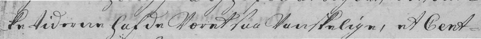ke tiderne hafde været saa Vanskelige, et Cent-
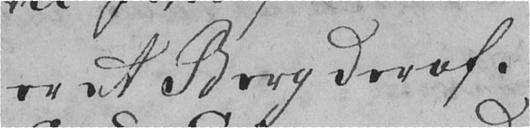er et Berg deraf.
